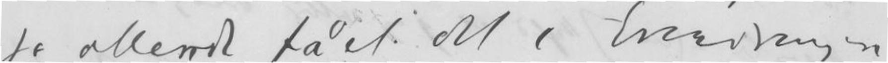jo allerede fået det i Erindringen
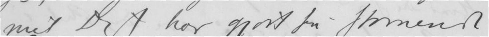mit Digt har gjort sin Stormende
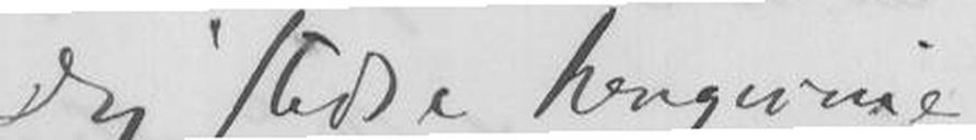Dig stedse hengivne
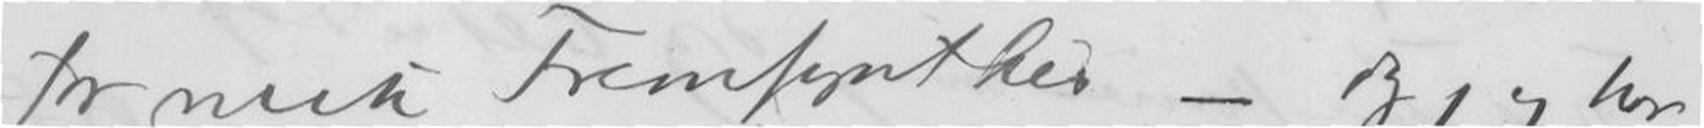for neste Fremsynhed - dog jeg har
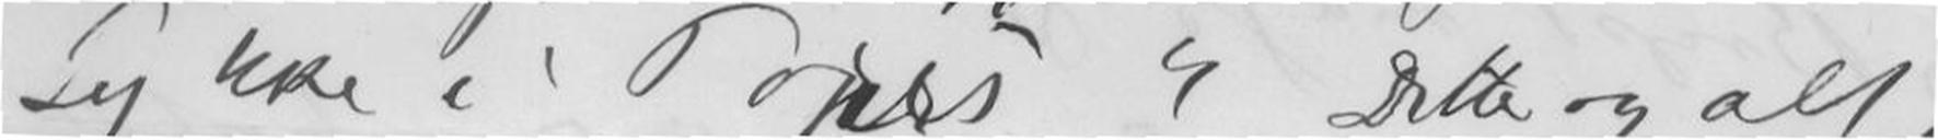Lykke i Paris " Dette og alt,
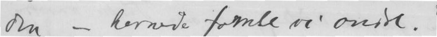den - hernede samler vi ondet".
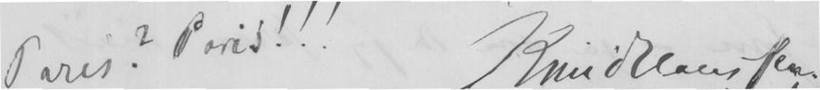Paris? Paris!!!Knud Claussen
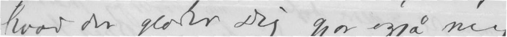hvad der glæder Dig gjør også mig
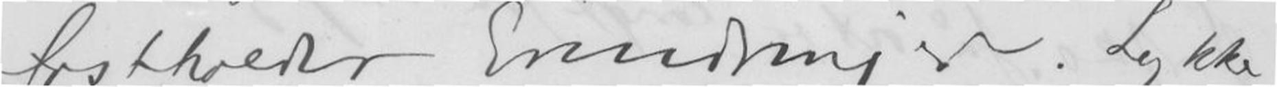fastholder Erindringer Lykke-
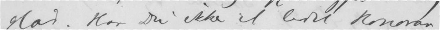glad. Har Du ikke et lidet Honorar
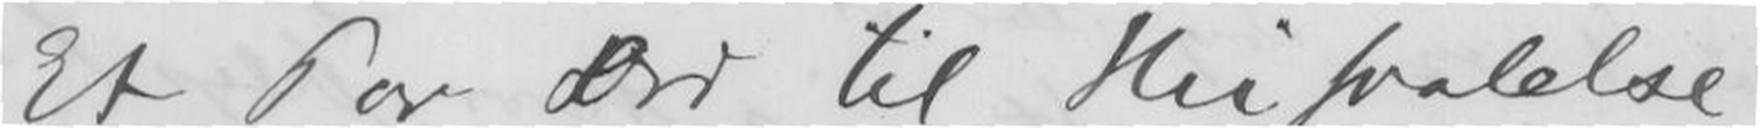Et Par Ord til Husvalelse
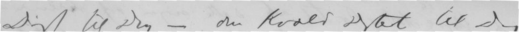Digt til Dig - den Kveld Digtet til Dig
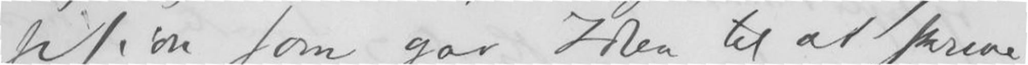sition som gav ieden til at skrive
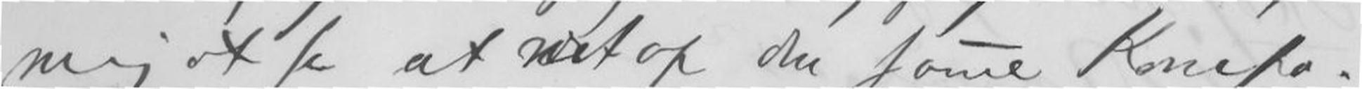mig at se at netop den same Kompo-
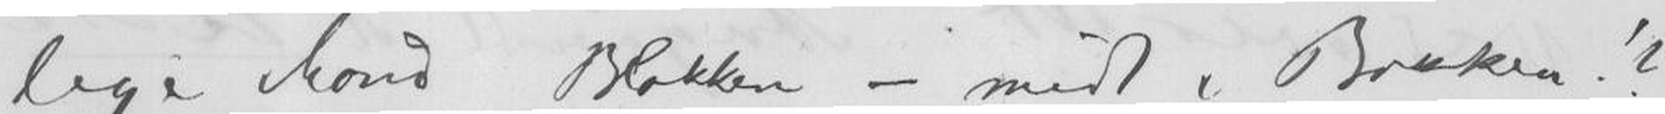lige Mand "Blakken - midt i Bakken!?
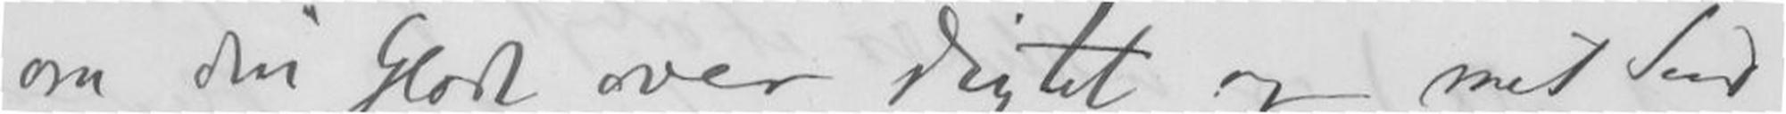om din Glæde over Digtet og mit Sind
